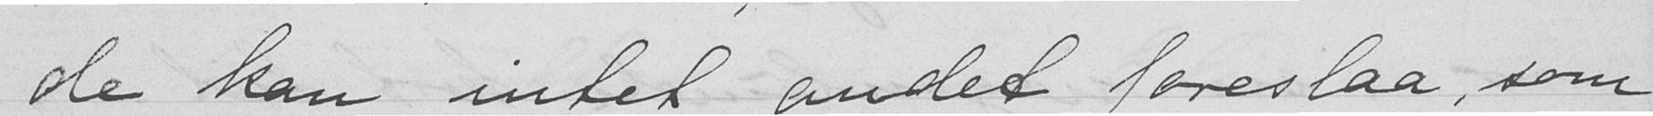de kan intet andet foreslaa, som
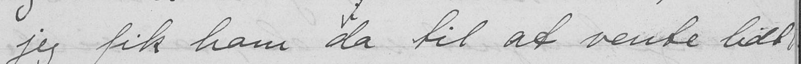jeg fik ham da til at vente lidt
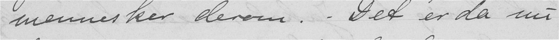mennesker derom. Det er da nu
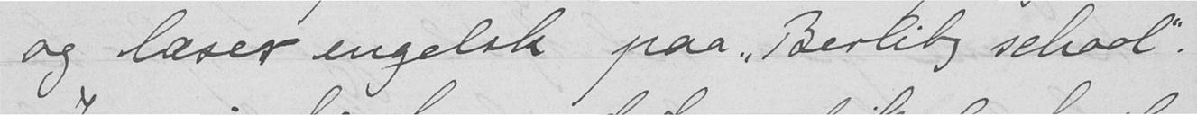og læser engelsk paa "Berlitz school".
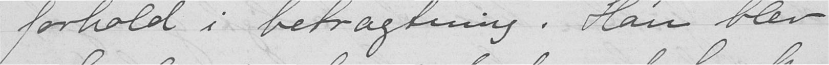forhold i betragtning. Han blev
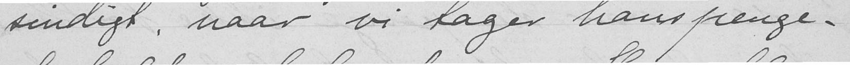sindigt, naar vi tager hans penge-
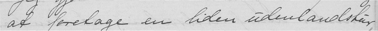at foretage en liden udenlandstur
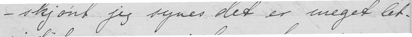- skjønt jeg synes det er meget let-
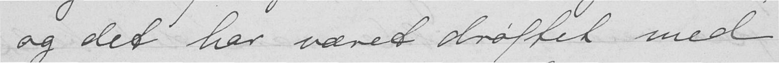og det har været drøftet med
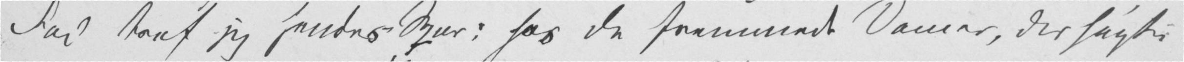Fod traf jeg hendes Spor: hos de fremmede Damer, der søgte
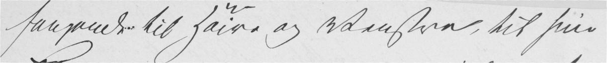fangende til Høire og Venstre, til sine
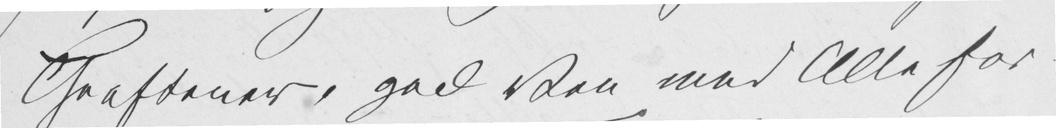Traftener, god Ven med Alle for-
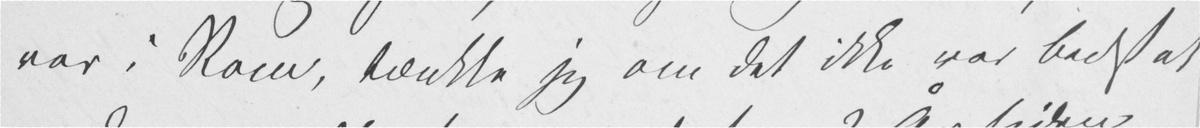var i Rom, tænkte jeg om det ikke var bedst at
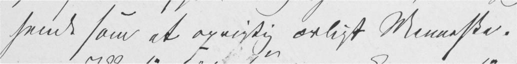hende som et oprigtig ærligt Menneske.
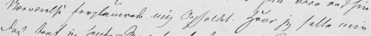Nærværelse forplumrede mig Opholdet. Hvor jeg satte min
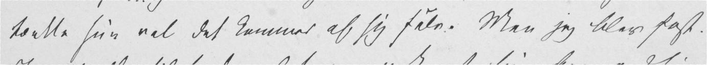tænkte hun vel det kommer af sig selv. Men jeg blev fast
